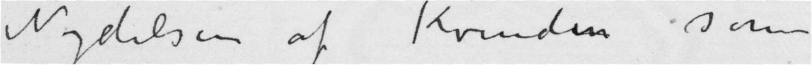Nydelsen af Kvinden som
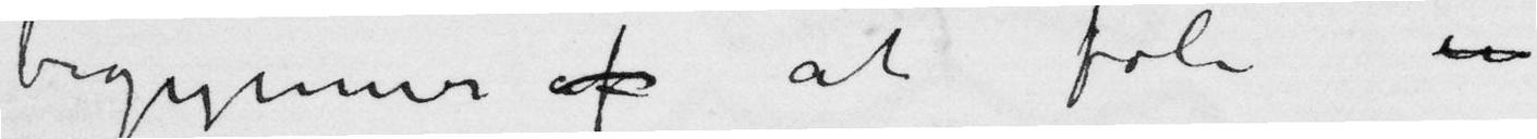begynner af at føle en
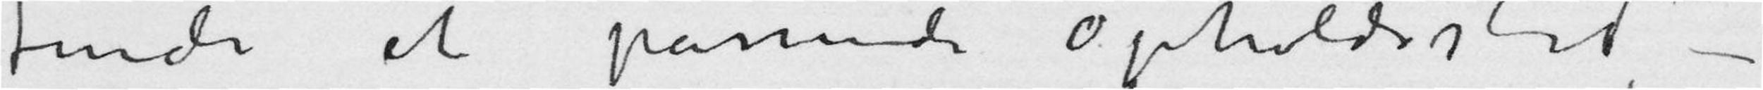finde et passende Opholdssted –
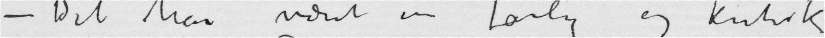– Det har været en farlig og kritisk
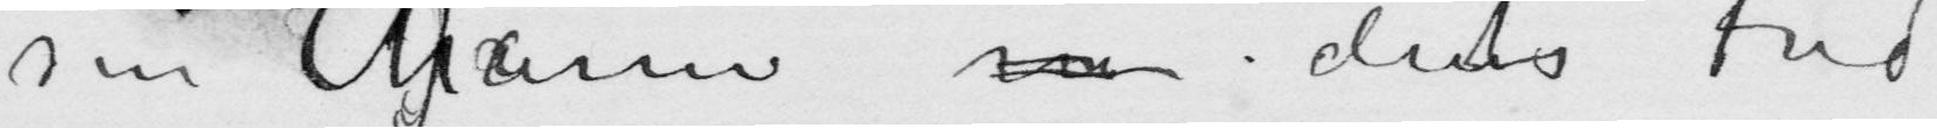sin Charmdents Fred
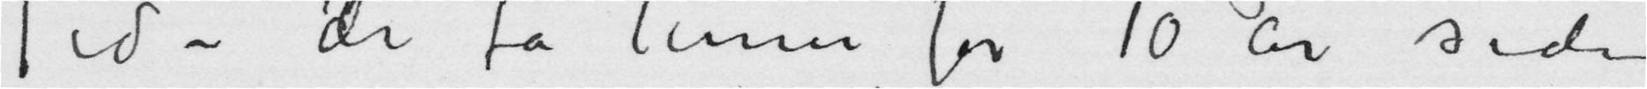Tid – De få Timer for 10 år siden
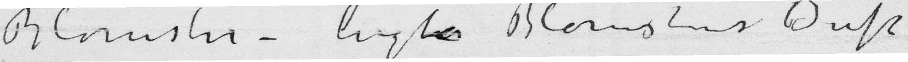Blomster – lugte Blomstens Duft
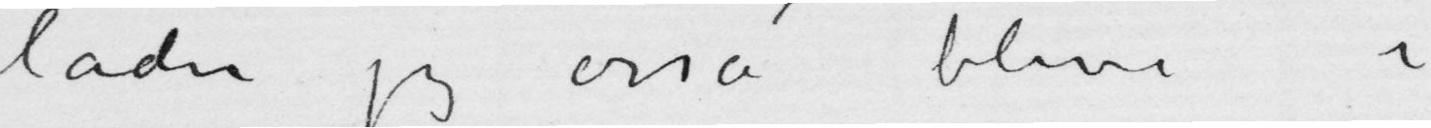lader jeg osså blive i
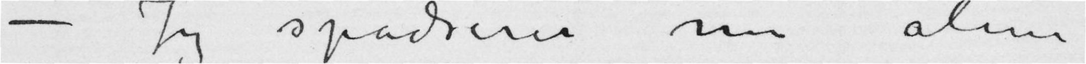– Jeg spadserer nu alene
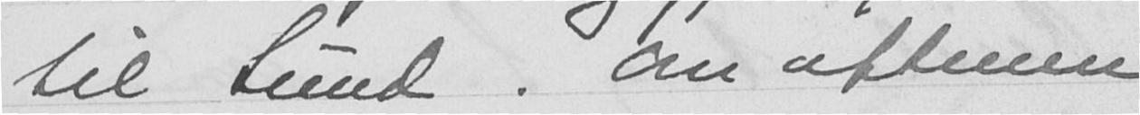til Sund. Om aftenen
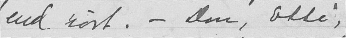end rørt. - Don, Etti,
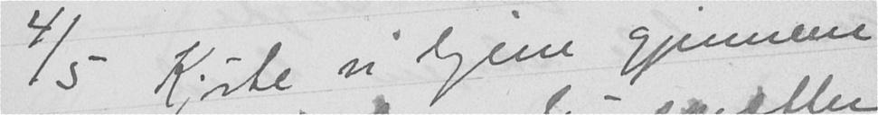4/5 - Kjørte vi hjem gjennem
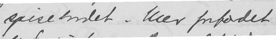spisebordet. Mer forfærdet
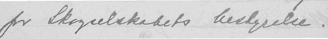for Skogselskabets bestyrelse.
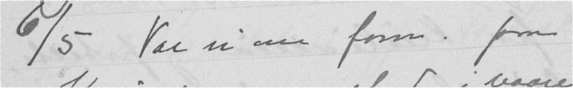6/5 Var vi om form. paa
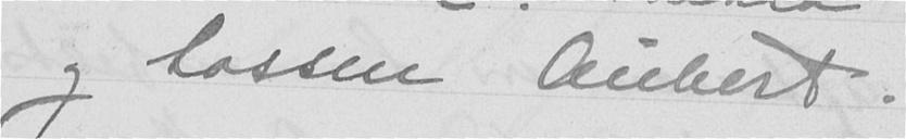og Lassen Aubert.
